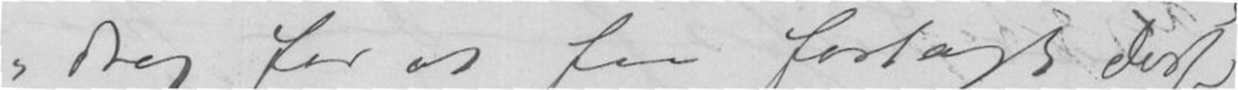drag for at faa forlagt disse
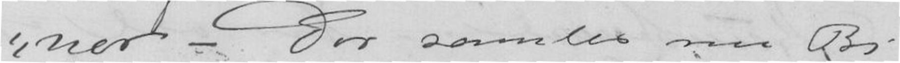ner - Der samles nu Bi
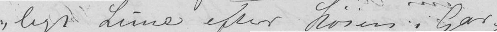ligt Lune efter Støien i Gar-
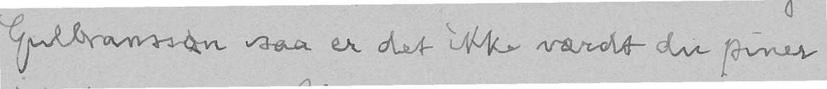Gulbransson saa er det ikke værdt du piner
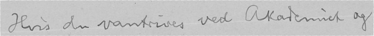Hvis du vantrives ved Akademiet og
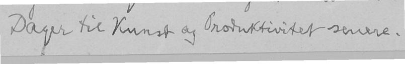Dager til Kunst og Produktivitet senere.
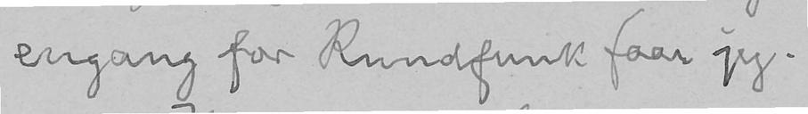engang for Rundfunk faar jeg.
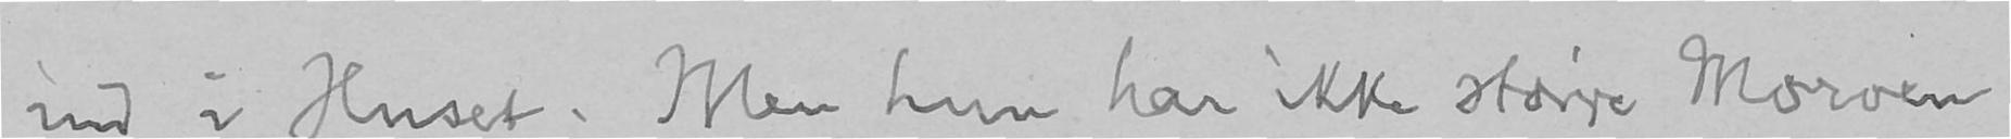ind i Huset. Men hun har ikke større Moroen
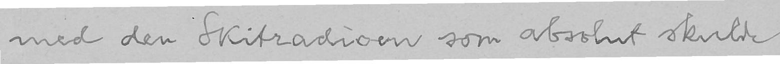med den Skitradioen som absolut skulde
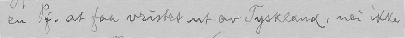en Pf. at faa vristet ut av Tyskland, nei ikke
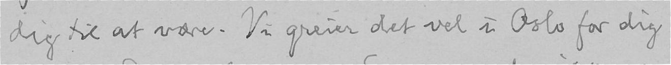dig til at være. Vi greier det vel i Oslo for dig
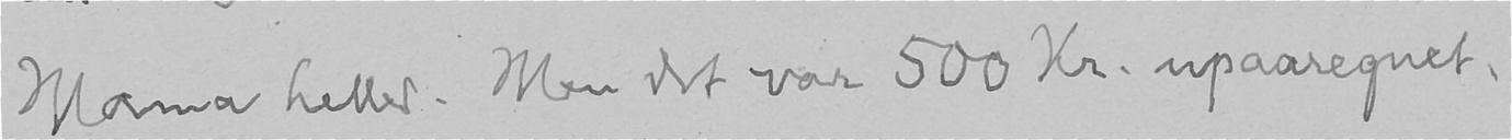Mama heller. Men det var 500 Kr. upaaregnet.
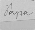Papa
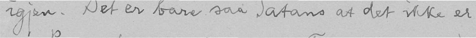igjen. Det er bare saa Satans at det ikke er
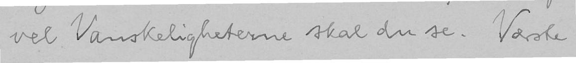vel Vanskeligheterne skal du se. Værste
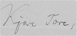Kjære Tore,
